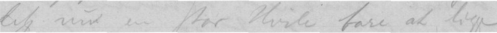det nu en stor Hvile bare at ligge
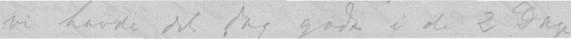vi havde det dog godt i de 2 Dage,
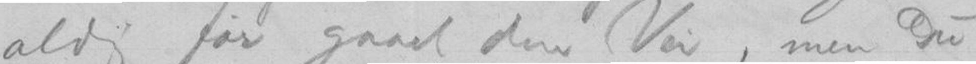aldrig før gaaet den Vei, men Du
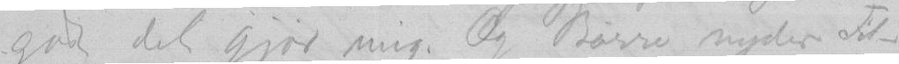godt det gjør mig. Og Børre nyder Til-
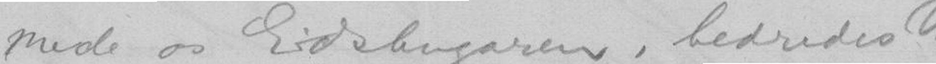mede os Eidsbugaren, bedredes Veiret,
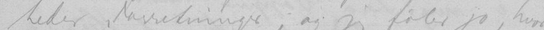heder Forretninger, og jeg føler jo, hvor
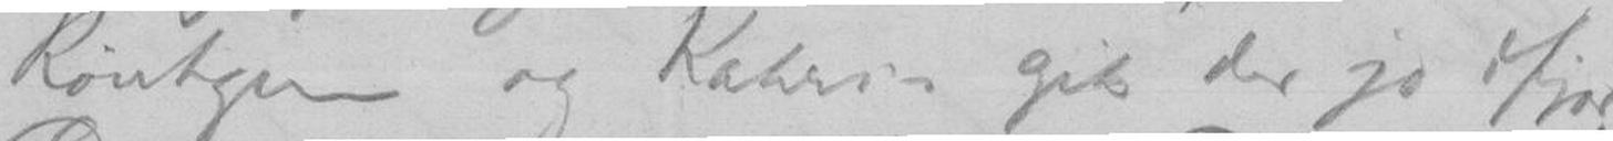Røntgen og Kahrs'n gik der jo ifjor.
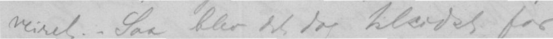veiret. - Saa blev det dog tilsidst for
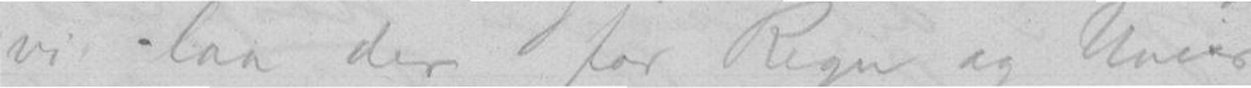vi laa der for Regn og Uveir.
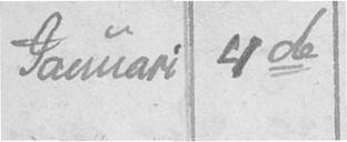Januari 4de
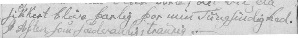sikkert blive farlig for min Tungsindighed.
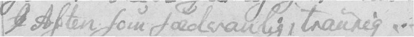I Aften som sædvanlig, traurig. –
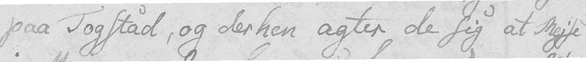paa Togstad, og derhen agter de sig at Rejse
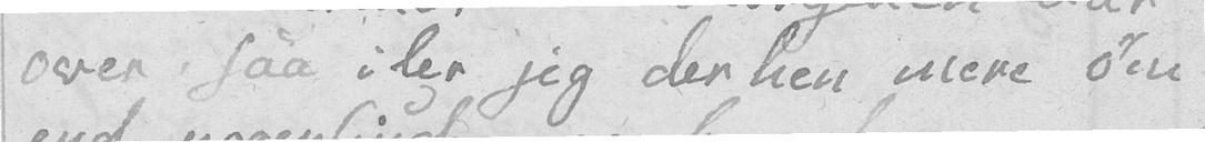over, saa iler jeg der hen mere øm
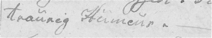traurig Humeur. –
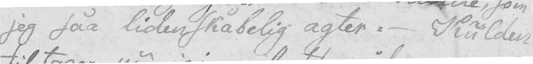jeg saa lidenskabelig agter. – Kulden
